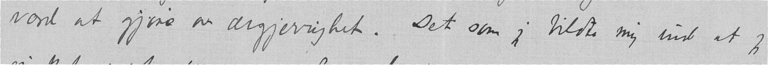værd at gjøre av ærgjerrighet. Det som jeg bildte mig ind at jeg
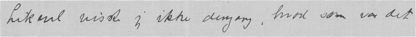Likevel visste jeg ikke dengang, hvad som var det
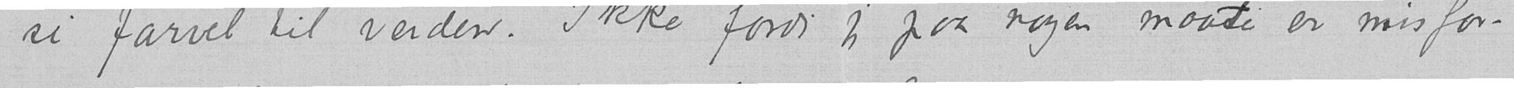si farvel til verden. Ikke fordi jeg paa nogen maate er misfor-
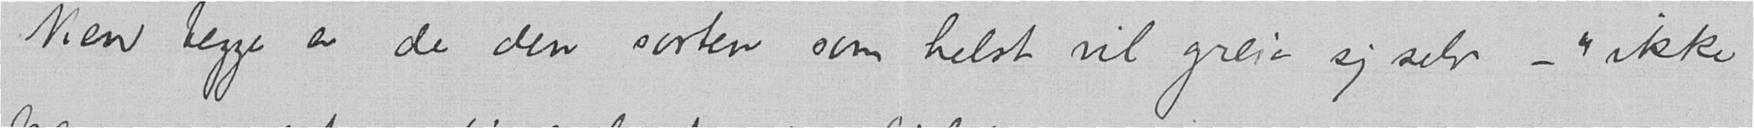Men begge er de den sorten som helst vil greie sig selv – "ikke
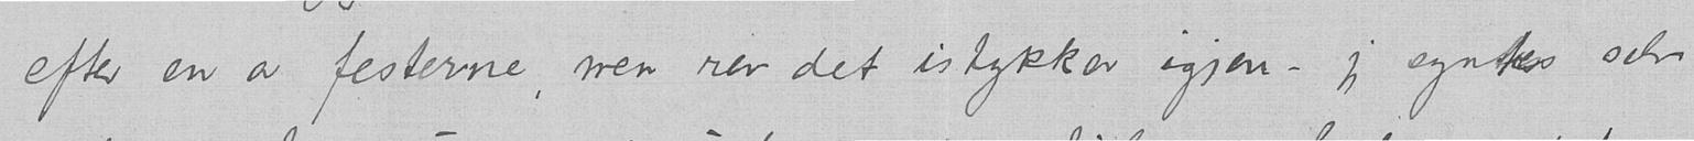efter en av festerne, men rev det istykker igjen – jeg syntes selv
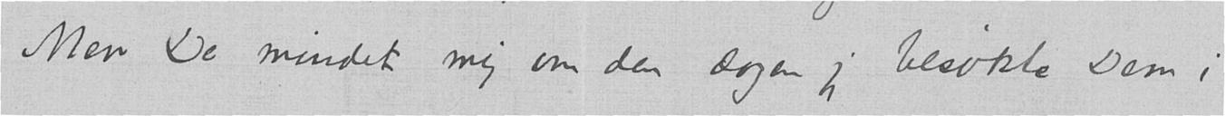Men De mindet mig om den dagen jeg besøkte Dem i
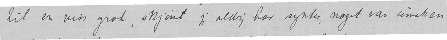til en viss grad, skjønt jeg aldrig har syntes, noget var umaken
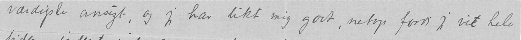værdigste ansigt, og jeg har likt mig godt, netop fordi jeg vet hele
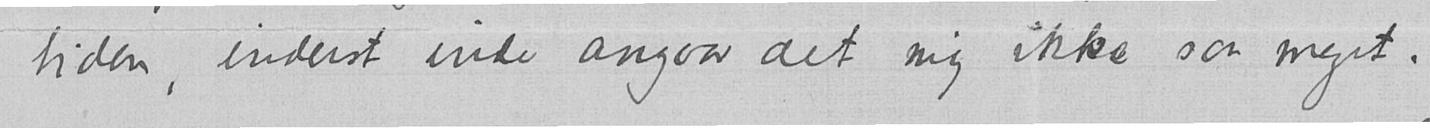tiden, inderst inde angaar det mig ikke saa meget.
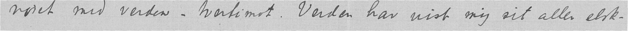nøiet med verden - tvertimot. Verden har vist mig sit aller elsk-
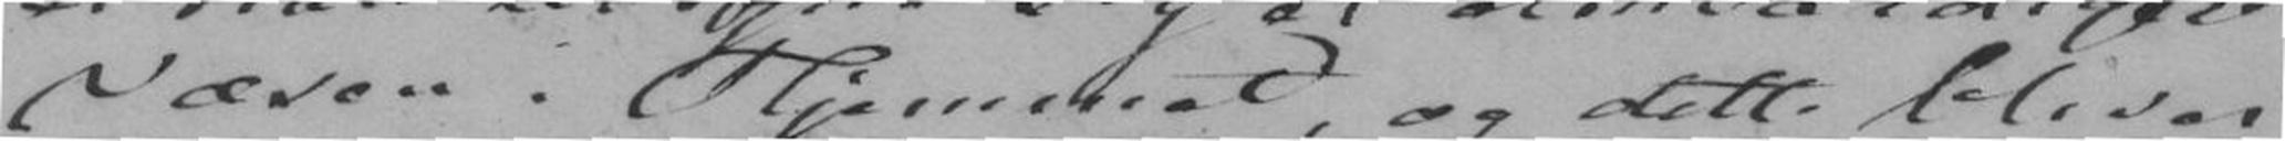Væsen i Hjemmet, og dette bliver
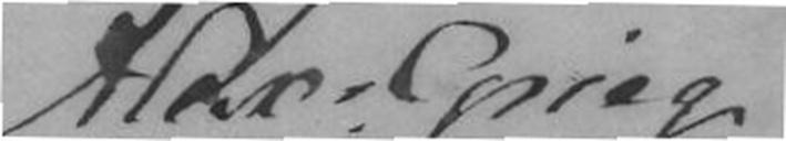Alexr. Grieg
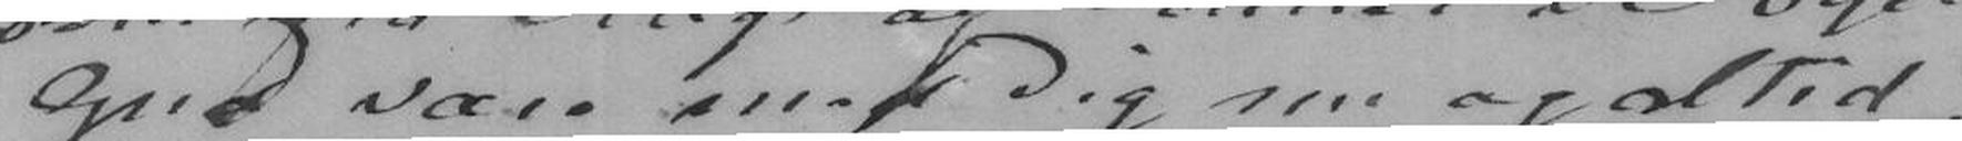Gud være med Dig nu og altid!
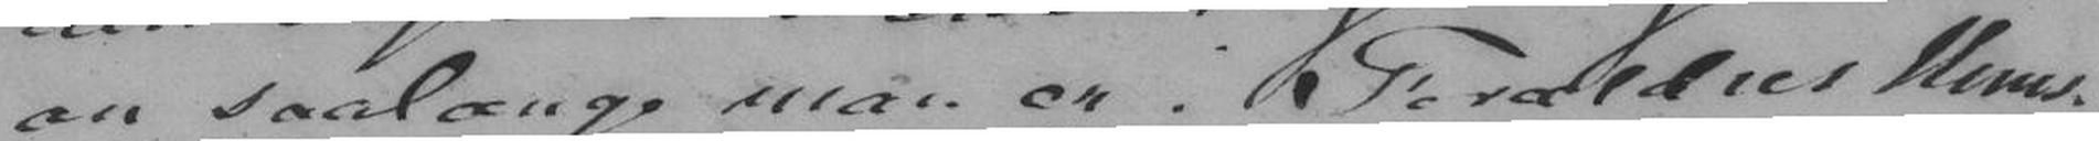an saalænge man er i Forældres Huus.
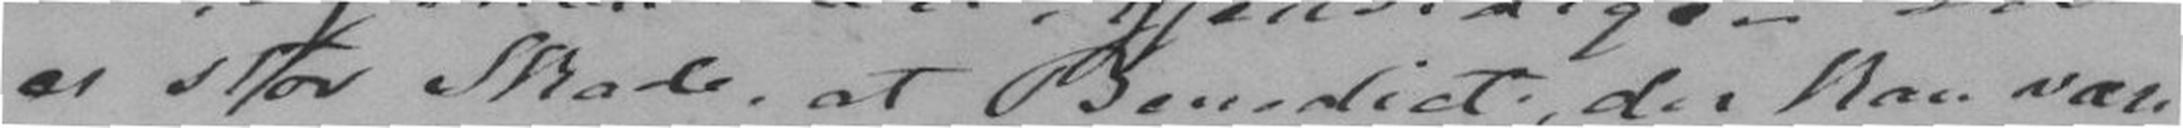er stor Skade, at Benedicte, der kan være
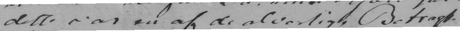dette var en af de alvorlige Betragt-
